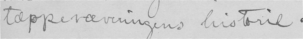tæppevævningens historie.
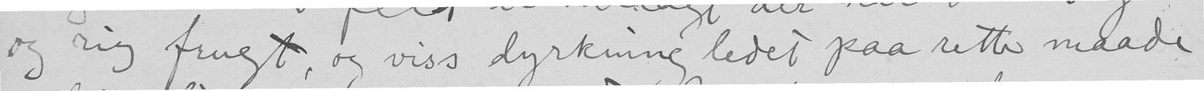og rig frugt, og viss dyrkning ledet paa rette maade
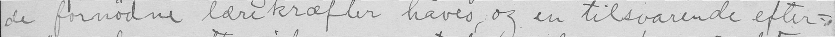de fornødne lærekræfter haves og en tilsvarende efter-
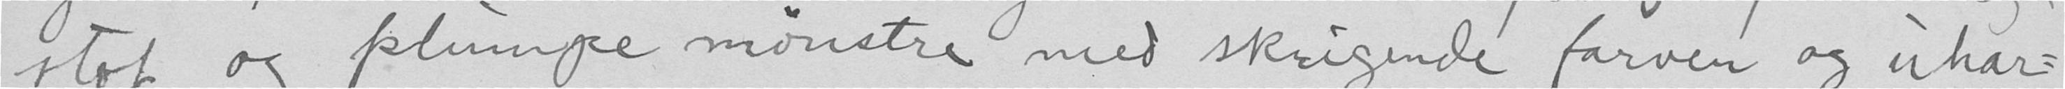stof og plumpe mønstre med skrigende farver og uhar-
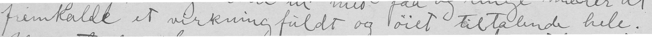fremkalde et virkningfuldt og øiet tiltalende hele.
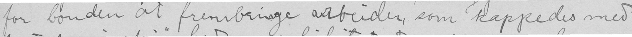for bonden at frembringe arbeider som kappedes med
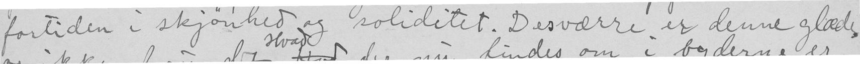fortiden i skjønhed og soliditet. Desværre er denne glæde
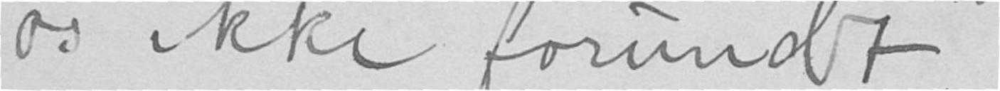os ikke forundt.
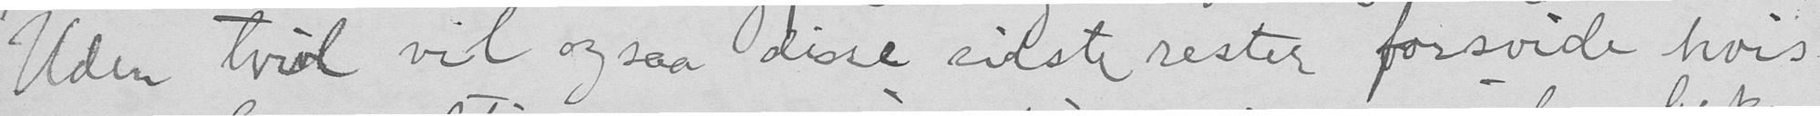Uden tvivl vil ogsaa disse sidste rester forsvide hvis
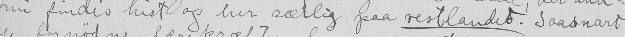nu findes hist og her særlig paa vestlandet. Saasnart
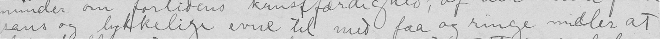sans og lykkelige evne til med faa og ringe midler at
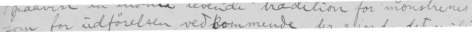paavise en endnu levende tradition for mønstrene
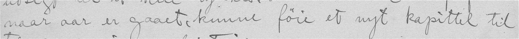naar aar er gaaet, kunne føre et nyt kapittel til
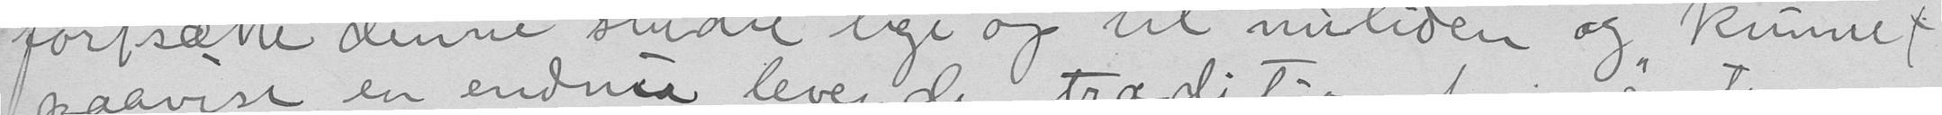fortsætte denne studie lige og til nutiden og kunnet
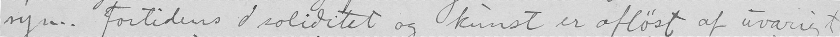syn. Fortidens  soliditet og kunst er aftøst af uvarigt
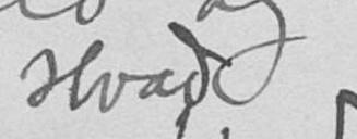Hvad
